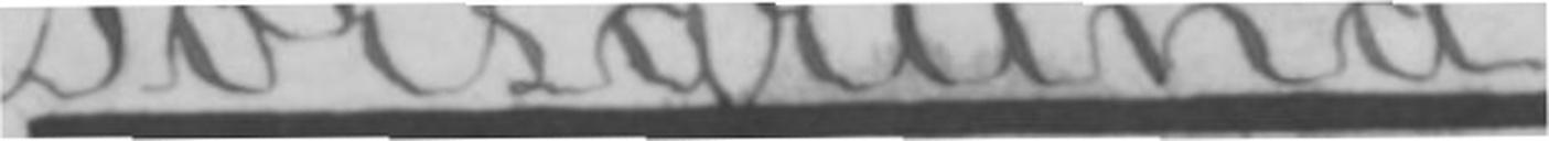Porsgrund.
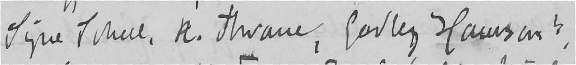Signe Scheel, R. Thrane, Godlef Hanson,
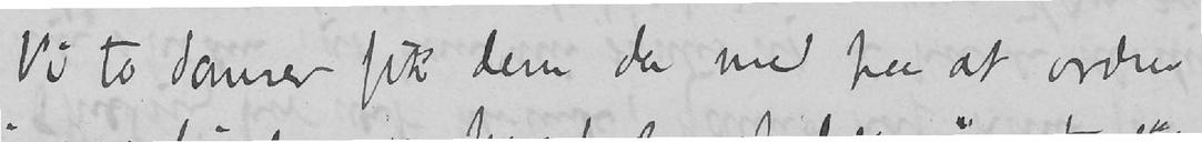Vi to damer fik dem da med paa at ordne
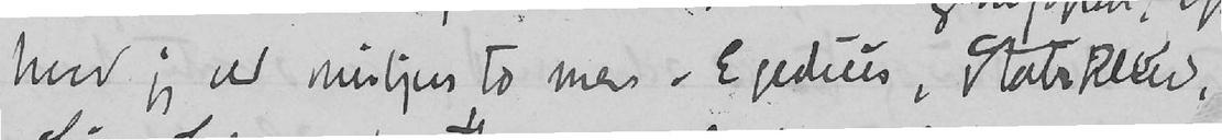hvad jeg ved muligens to mer, Egedius, Statskleiv,
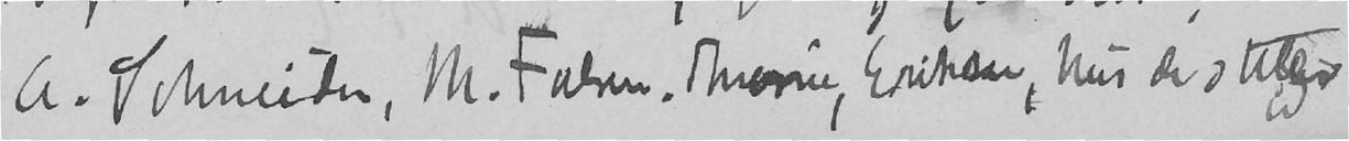A. Schneider, M. Falsen, Thorne, Eriksen, hvis de stiller
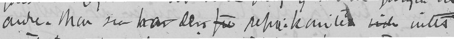andre. Men saa har den fu repr.komité inh intet
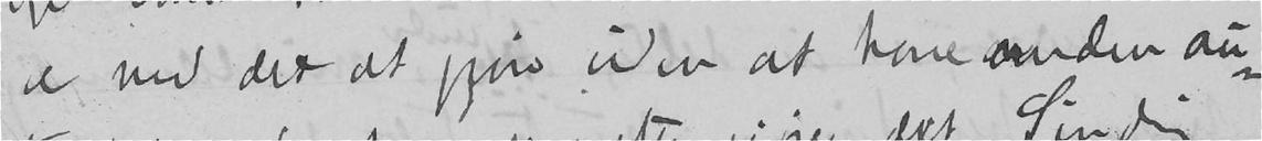ve med det at gjøre uden at have anden au-
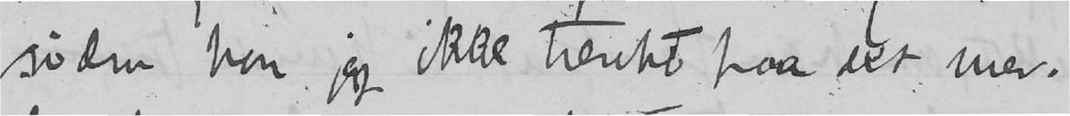siden har jeg ikke tænkt paa det mer.
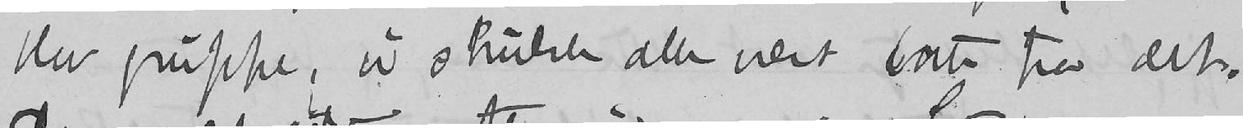blev gruppe, vi skulde alle vært borte fra det.
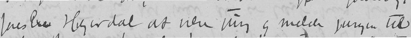foreslo Heyerdal at være jury og melde juryen til
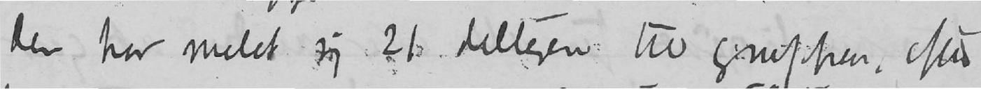Der har meldt sig 21 deltagere til gruppen, efter
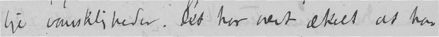lige vanskeligheder. Det har vært ækelt at ha-
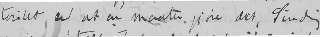toritet end, at en maatte gjøre det, Sinding
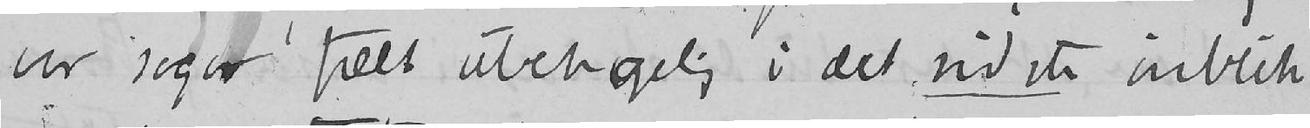var sogar fælt ubehagelig i det sidste øieblik
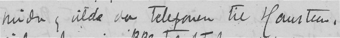kuelse og vilde da telefonere til Hansteen,
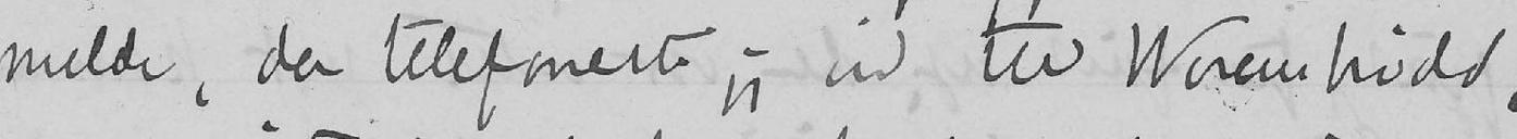melde, da telefonerete jeg ud til Werenskiold,
